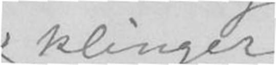klinger,
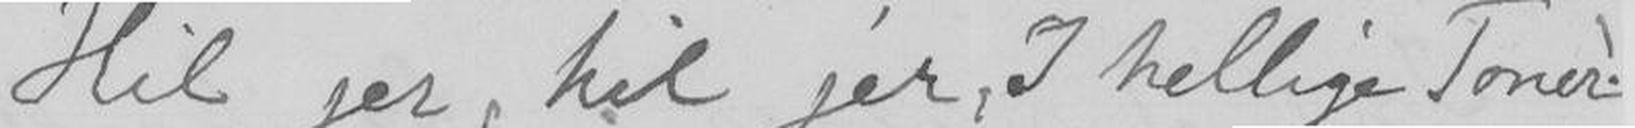Hil jer, hil jer, I hellige Toner.
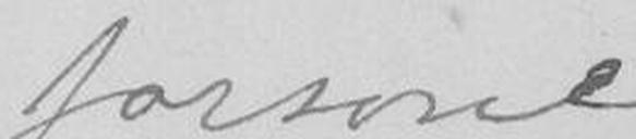forsone.
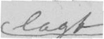lagt,
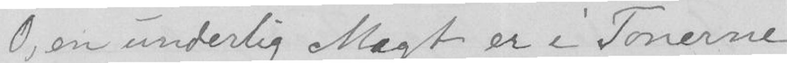O, en underlig Magt er i Tonerne
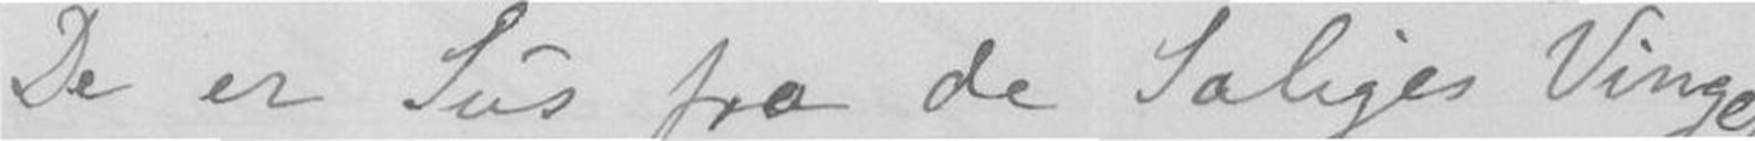De er Sus fra de Saliges Vinger,
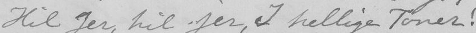Hil jer, hil jer, I hellige Toner!
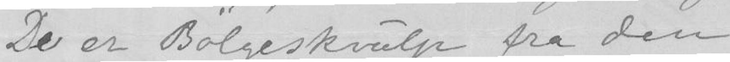De er Bølgeskvulp fra den
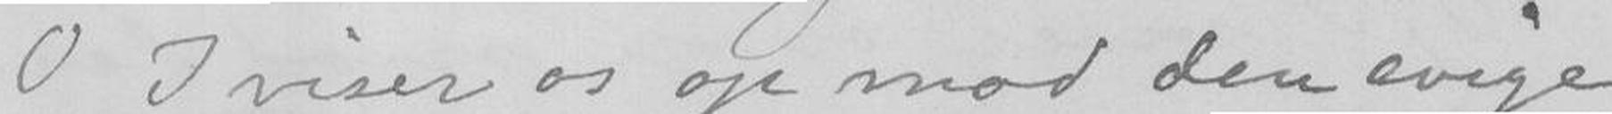O I viser os op mod den evige
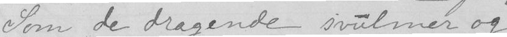Som de dragende svulmer og
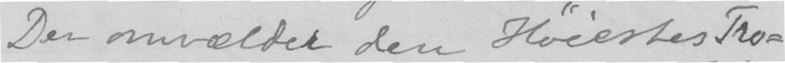Der omvælder den Høiestes Tro-
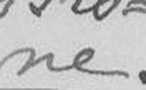ne;
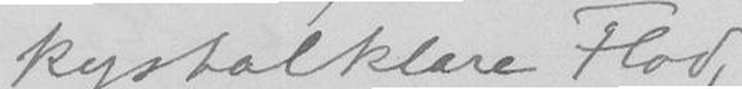kystalklare Flod,
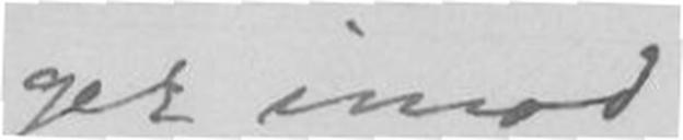ger imod
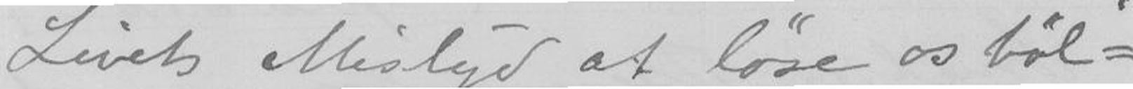Livets Mislyd et løse os bøl-
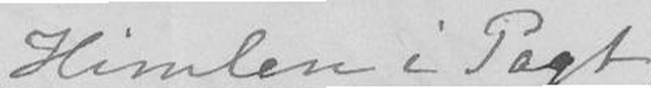Himlen i Pagt,
